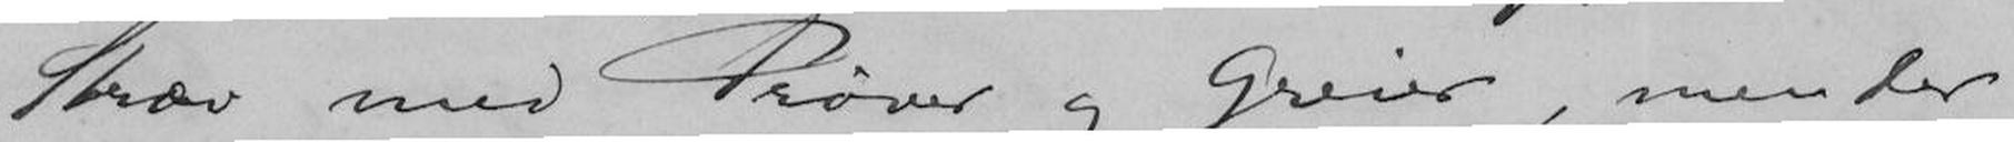Stræv med Prøver og Greier, men der
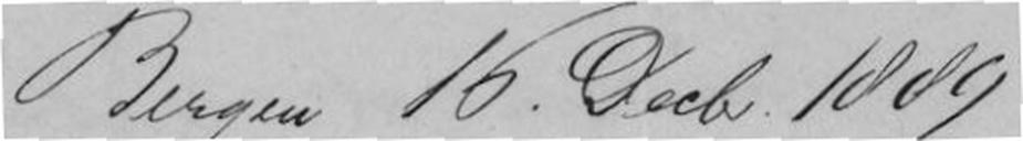Bergen 16 Decb. 1889
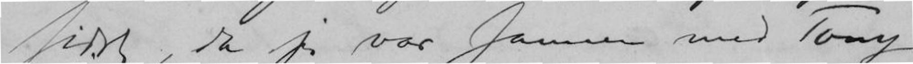sidst, da jeg var sammen med Tony,
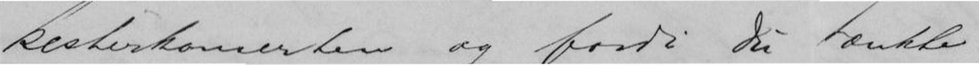kesterkonserten og fordi Du tænkte
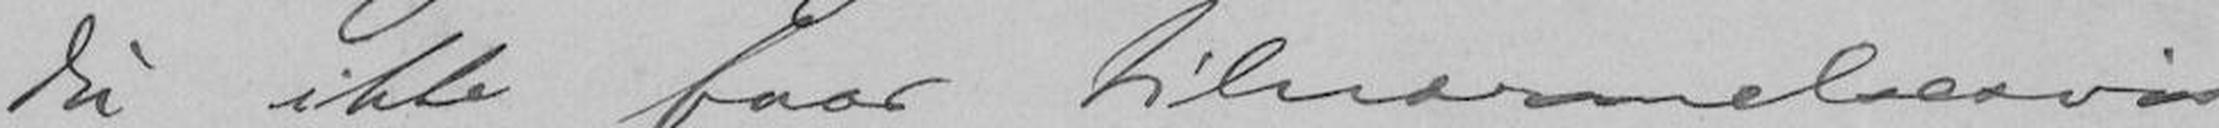du ikke faar tilnærmelsesvis
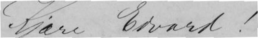Kjære Edvard!
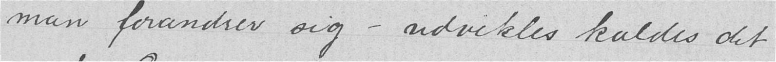man forandrer sig - udvikles kaldes det
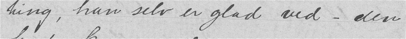ting, han selv er glad ved - den
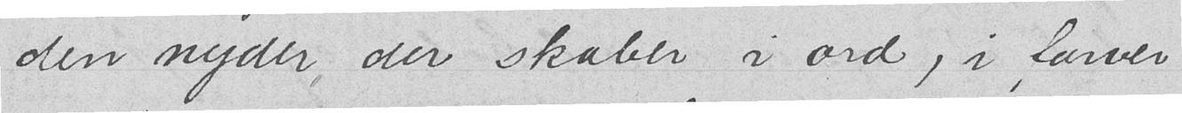den nyder, der skaber i ord, i farver
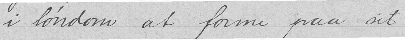i løndom at forme paa sit
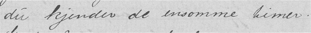du kjender de ensomme timer.
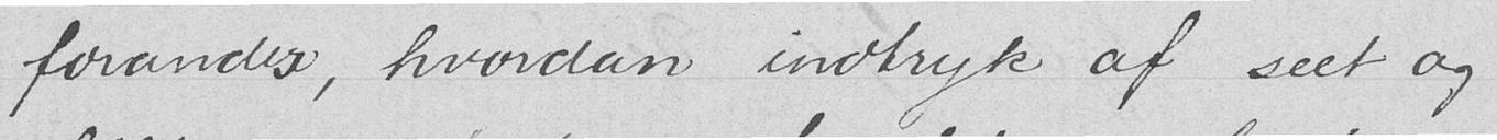forandres, hvordan indtryk af seet og
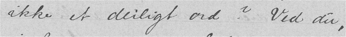ikke et deiligt ord? Ved du,
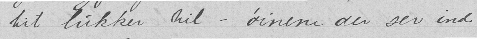tit lukket til – øinene der ser ind
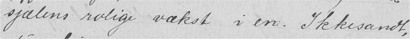sjælens rolige vækst i en. Ikkesandt,
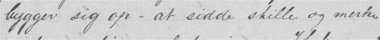bygger sig op - at sidde stille og merke
